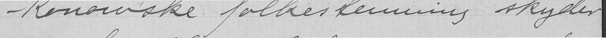-Konowske folkestemming skyder
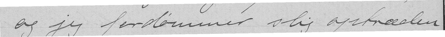og jeg fordømmer slig optræden
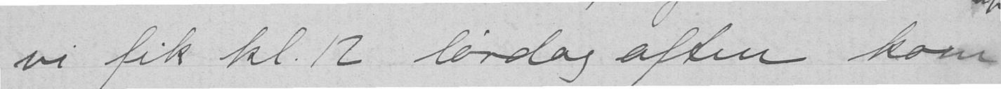vi fik kl. 12 lørdag aften kom
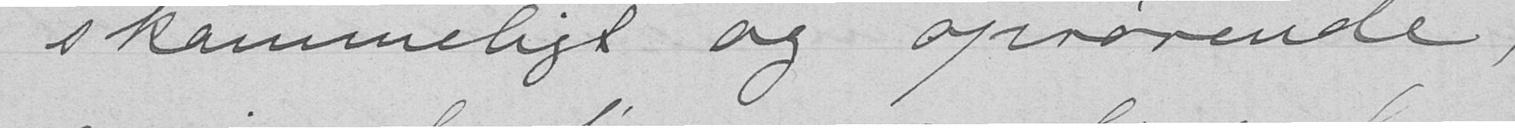skammeligt og oprørende,
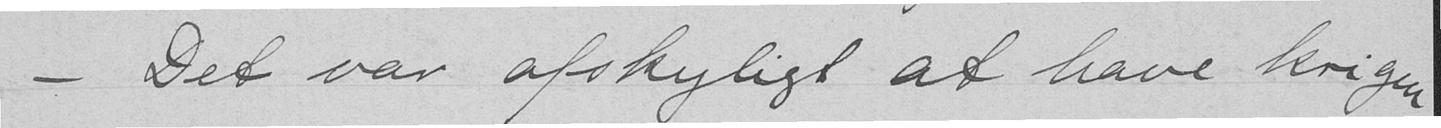- Det var afskyligt at have krigen
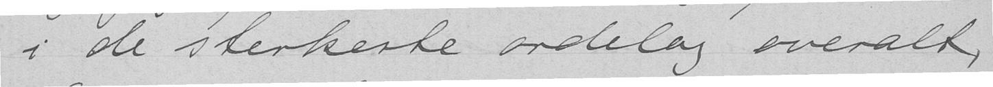i de sterkeste ordelag overalt,
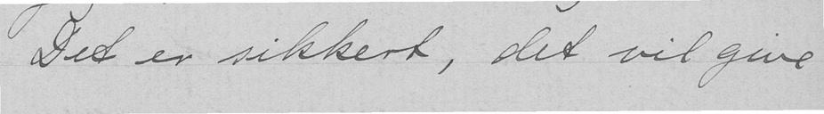Det er sikkert, det vil give
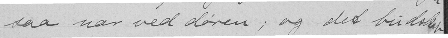saa nær ved døren; og det budskab
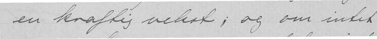en kraftig vekst; og om intet
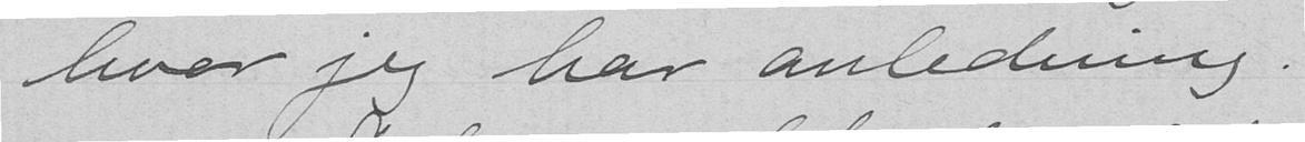hvor jeg har anledning.
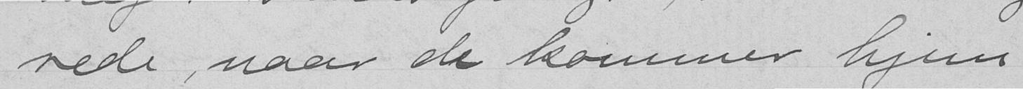rede, naar de kommer hjem
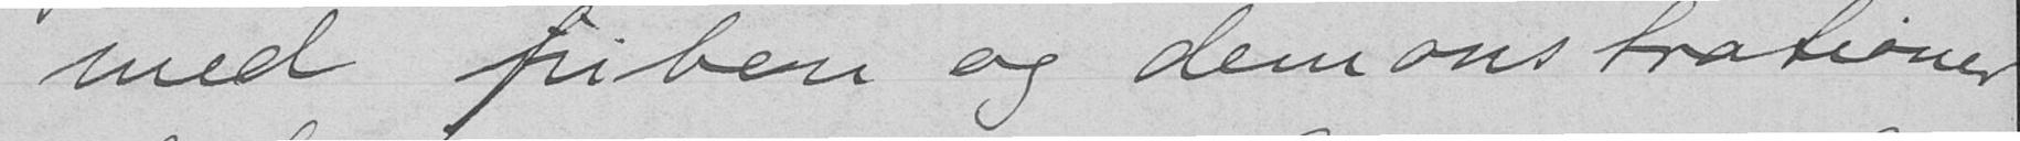med piben og demonstrationer
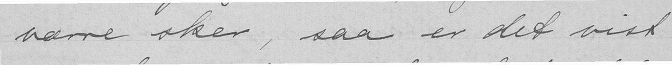værre sker, saa er det vist
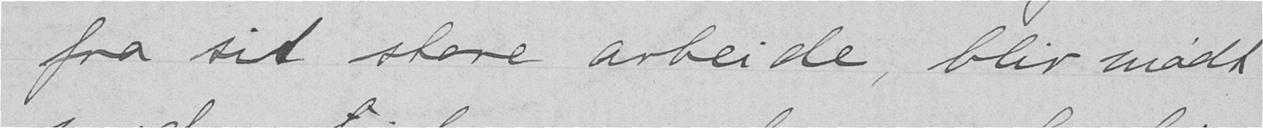fra sit store arbeide, blir mødt
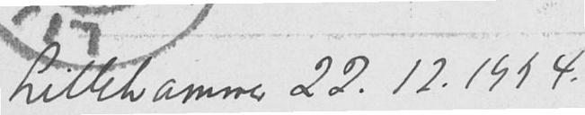Lillehammer 22.12.1914
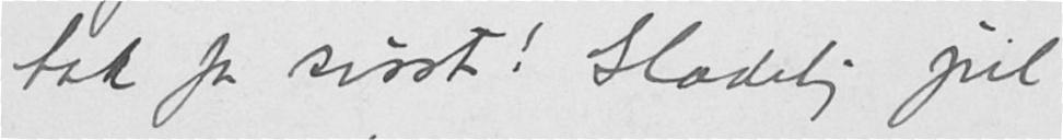tak for sisst! Glædelig jul
